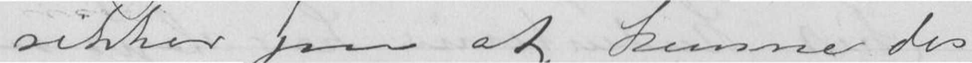sikker paa at kunne dis-
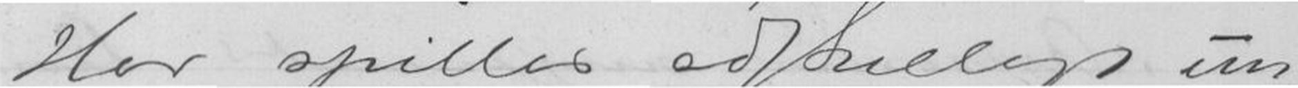Her spilles adskilligt un-
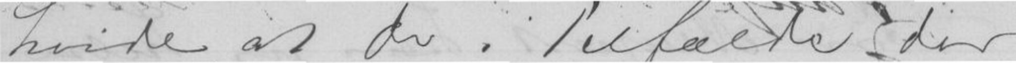hvide at De i Tilfælde der
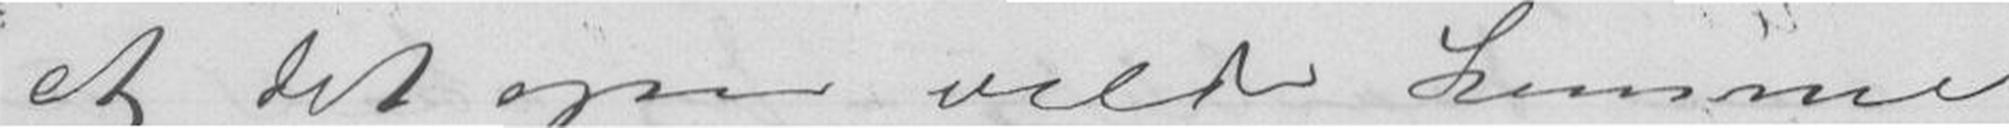at det ogsaa vilde komme
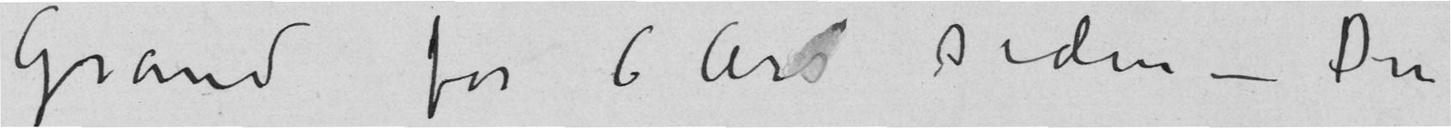Grand for 6 år siden – Det
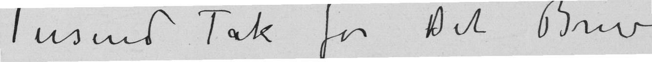Tusind Tak for Dit Brev.
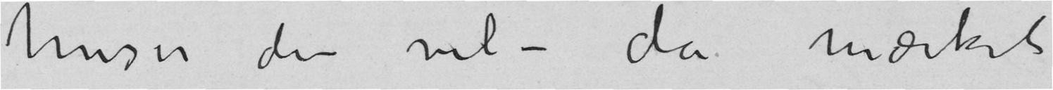husker du vel – da mærket
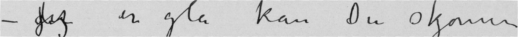– Jeg er gla kan du skjønne
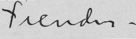Fiender.
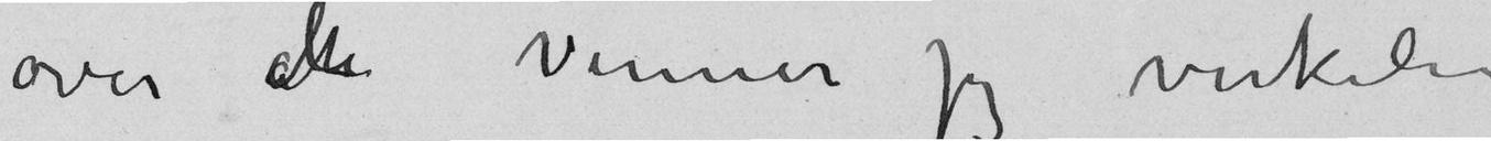over de venner jeg virkelig
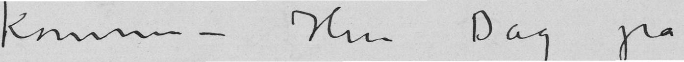komme – Hin Dag på
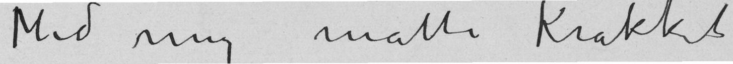Med mig måtte Krakket
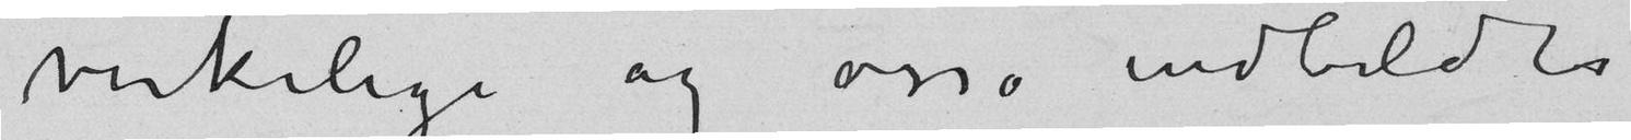virkelige og osså indbildte
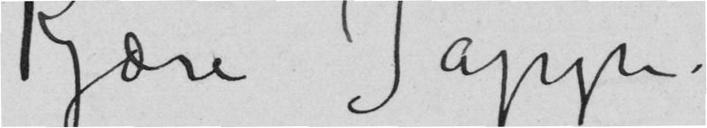Kjære Jappe.
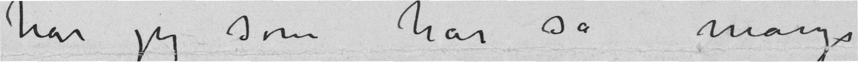har jeg som har så mange
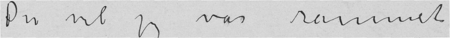Du vel jeg var rammet
